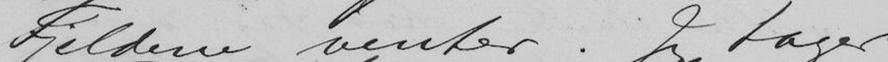Fjeldene venter. Jeg tager
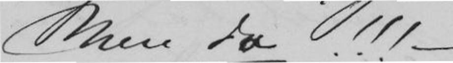Men da!!!
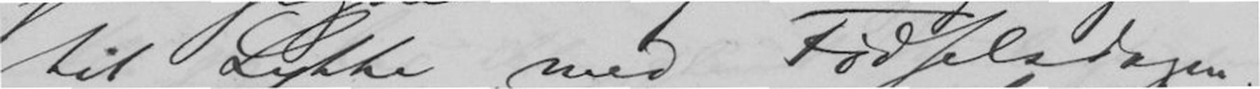til Lykke med Fødselsdagen!
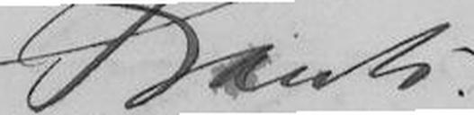Frants.
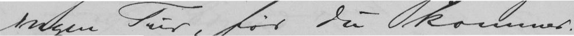ingen Tur, før du kommer.
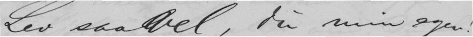Lev saa vel, du min egen!
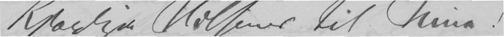Kjærligste Hilsener til Nina!
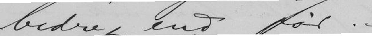bedre end før.-
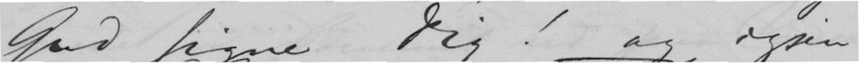Gud signe dig! og igjen
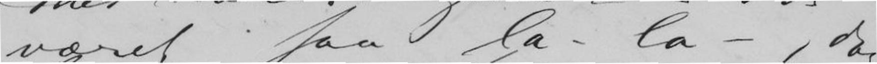været saa la - la -, dog
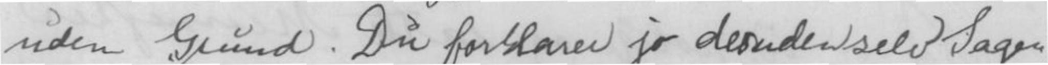uden Grund. Du forklarer jo desuden selv Sagen,
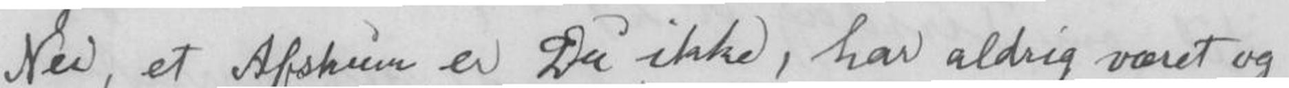Nei, et Afskum er Du ikke, har aldrig været og
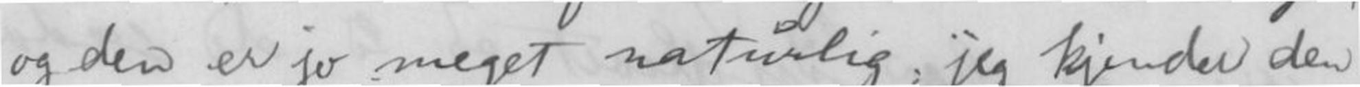og den er jo meget naturlig; jeg kjender den
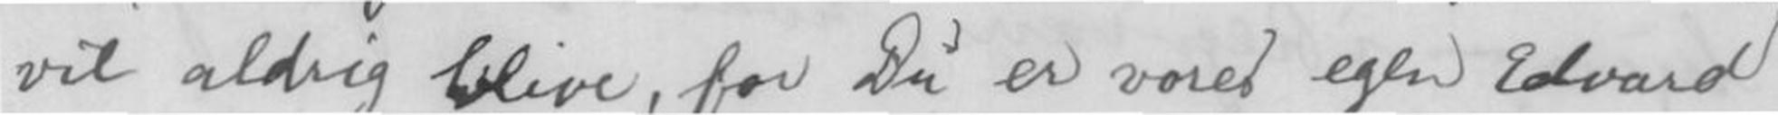vil aldrig blive, for Du er vores egen Edvard
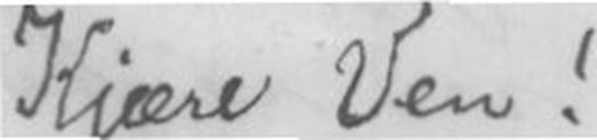Kjære Ven!
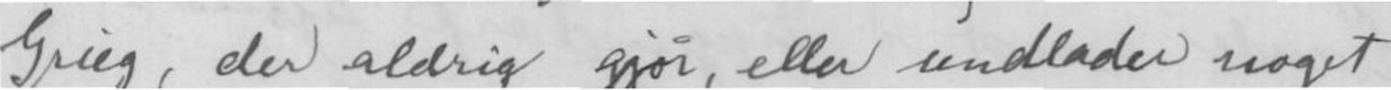Grieg, der aldrig gjør, eller undlader noget
